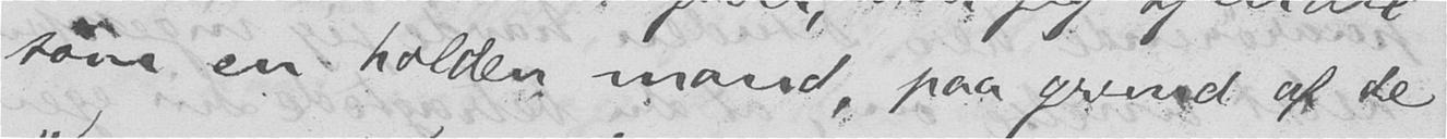som en holden mand, paa grund af de
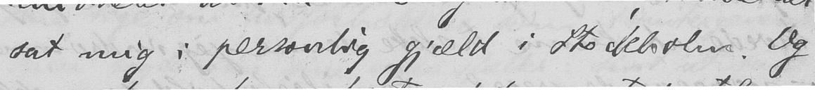sat mig i personlig gjæld i Stockholm. Og
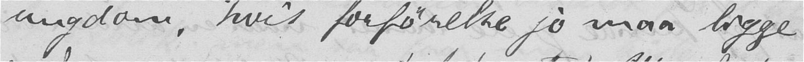ungdom, hvis forførelse jo maa ligge
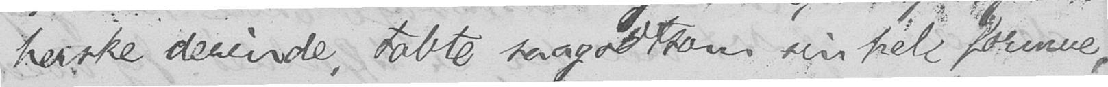herske derinde, tabte saagodtsom sin hele formue,
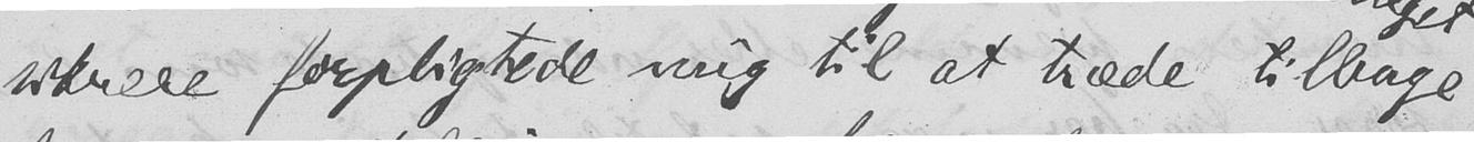sikrere forpligtede mig til at træde tilbage
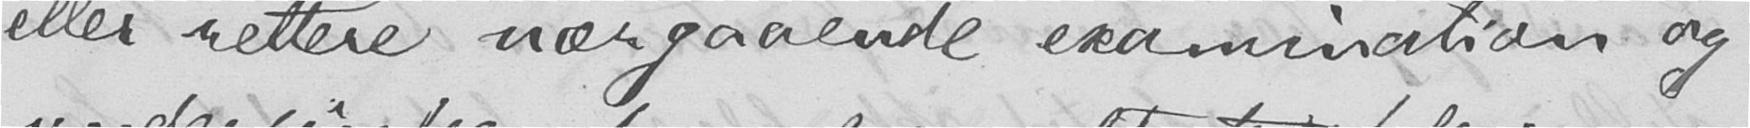eller rettere nærgaaende examination og
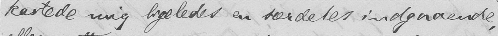kastede mig ligeledes en særdeles indgaaende,
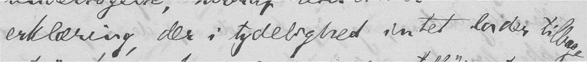erklæring, der i tydelighed intet lader tilbage
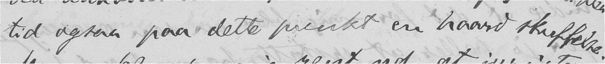tid ogsaa paa dette punkt en haard skuffelse.
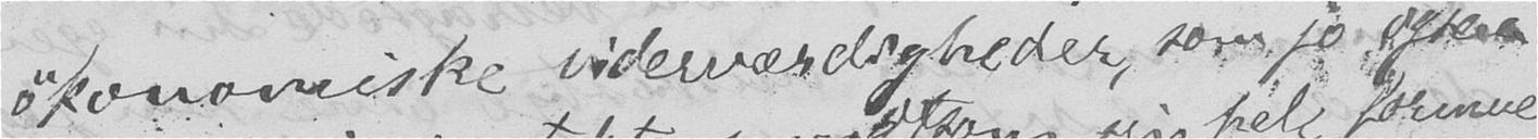økonomiske viderværdigheder, som jo ogaa
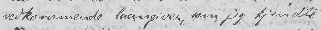vedkommende laangiver, som jeg kjendte
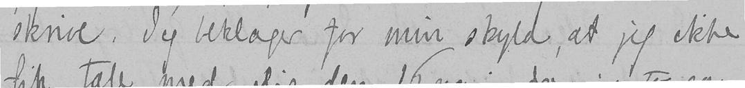skrive. Jeg beklager for min skyld, at jeg ikke
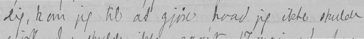Dig, kom jeg til at gjøre, hvad jeg ikke skulde
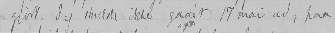gjort. Jeg skulde ikke gaaet 17 mai ud; paa
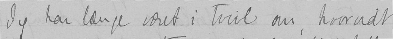Jeg har længe været i tvivl om, hvorvidt
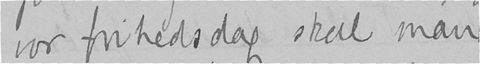vor frihedsdag skal man
